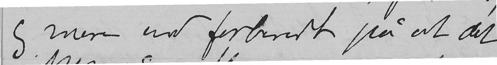og mere end forberedt på at det
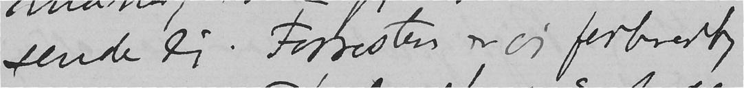sende Dig. Forresten er jeg forberedt
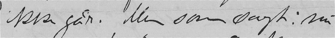ikke går. Men som sagt: nu
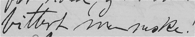bittert mennske!
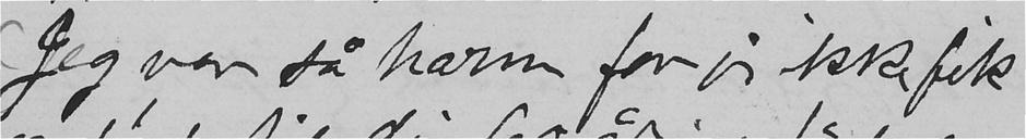Jeg var så harm for jeg ikke fik
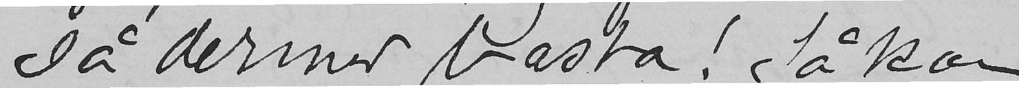så dermed basta! Så kan
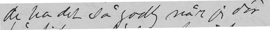de ha det så godt når jeg dør
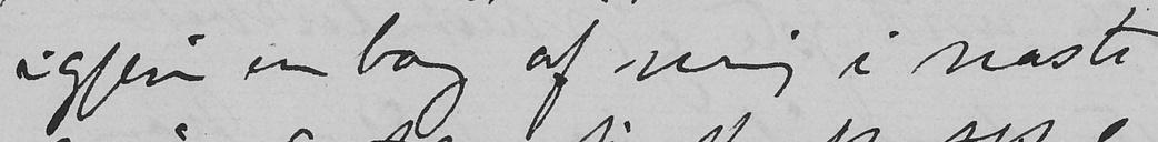igjen en bog af mig i neste
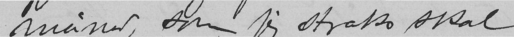måned, som jeg straks skal
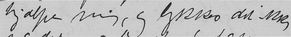hjælpe mig, og lykkes det ikke,
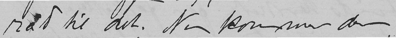råd til det. Nu kommer der
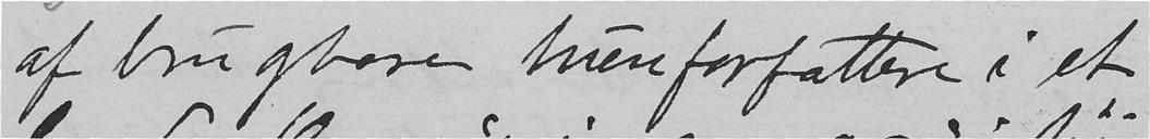af brugbare hunforfattere i et
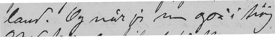land. Og når jeg nu også i høj
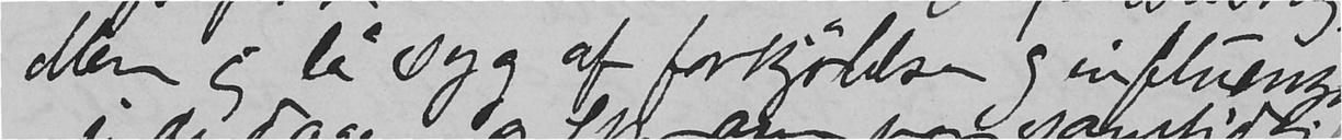Men jeg lå syg af forkjølelse og influensa
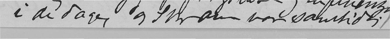i de dager, og Skram var samtidlig
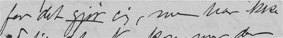for det gjør jeg, nu har ikke
